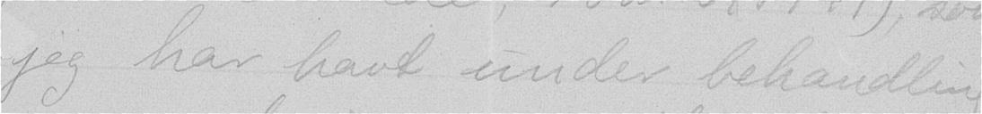jeg har havt under behandling
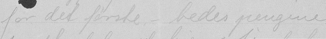for det første bedes pengene
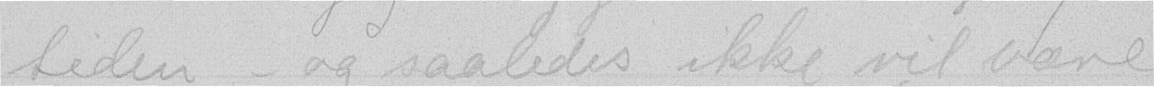tiden ,- og saaledes ikke vil være
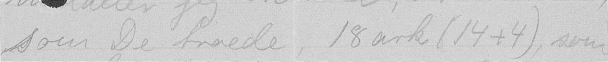som De troede, 18 ark (14+4) som
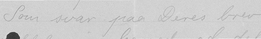Som svar paa Deres brev
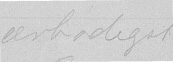ærbødigst
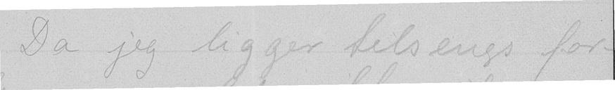Da jeg ligger tilsengs for-
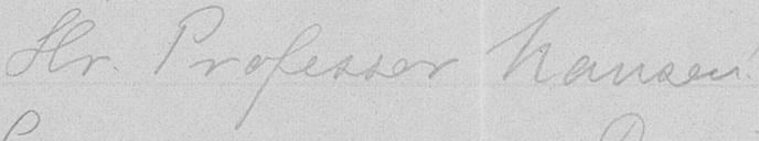Hr. Profestor Hansen:
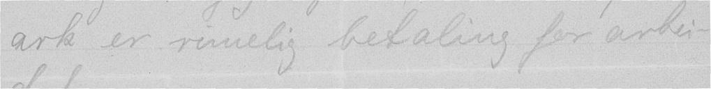ark er rimelig betaling for arbei-
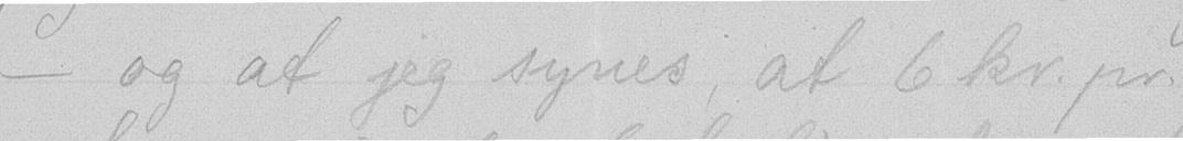og at jeg synes at 6kr. pr.
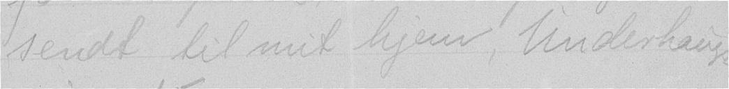sendt til mit hjem, Underhaug
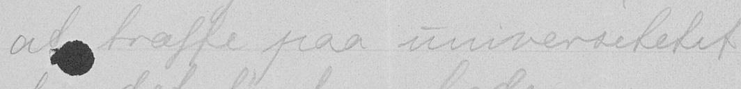at træffe paa universitetet
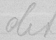det
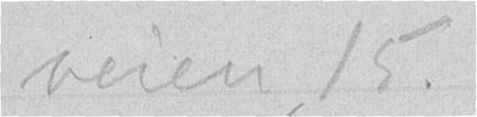veien 15.
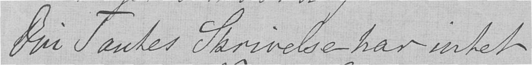Din Tantes Skrivelse har intet
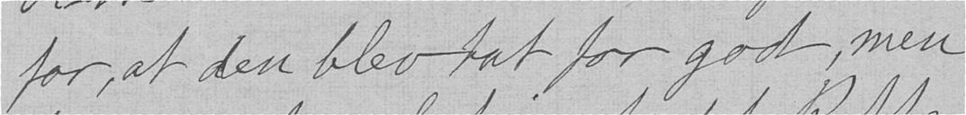for, at den blev tat for godt, men
